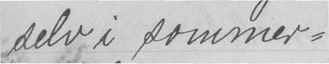selv i sommer-
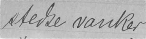stedse vanker
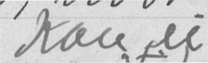Kan ei
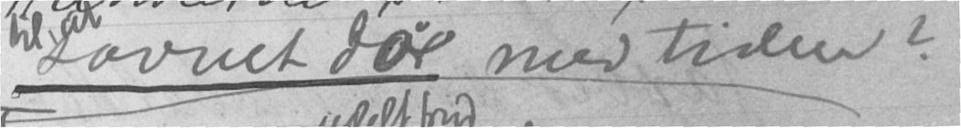savnet dør med tiden?
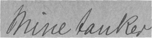Mine tanker
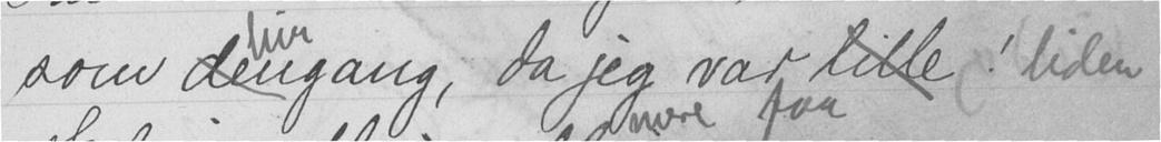som denhingang, da jeg var lille! liden
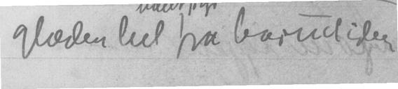glæden hel fra barnetiden
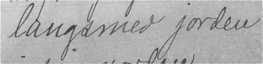langsmed jorden
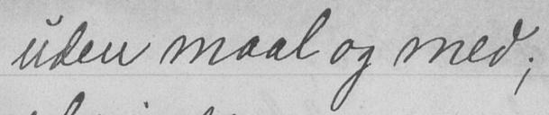uden maal og med;
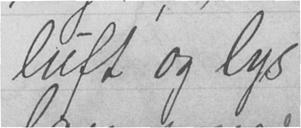lult og lys
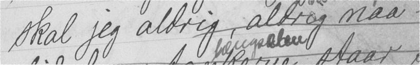skal jeg aldrig, aldrig naa
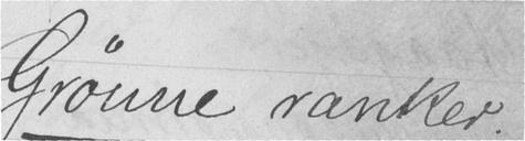Grønne ranker.
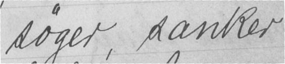søger, sanker
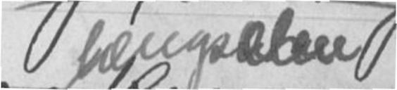længselen
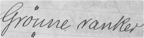Grønne ranker
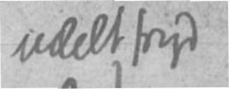udelt fryd
